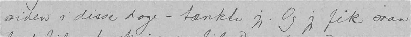siden i disse dage - tænkte jeg. Og jeg fik saan
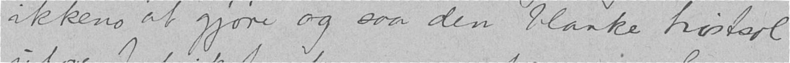ikkeno at gjøre og saa den blanke høstsol
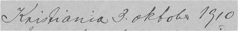Kristiania 3. oktober 1910
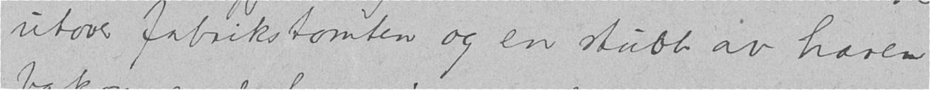utover fabrikstomten og en stubb av haven
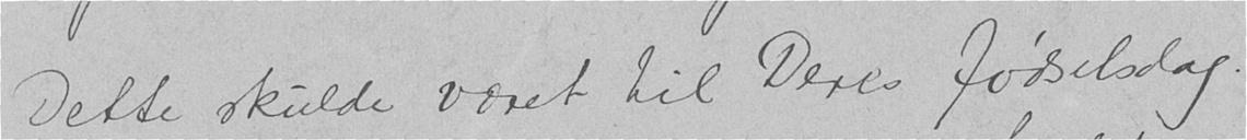Dette skulde været til Deres fødselsdag.
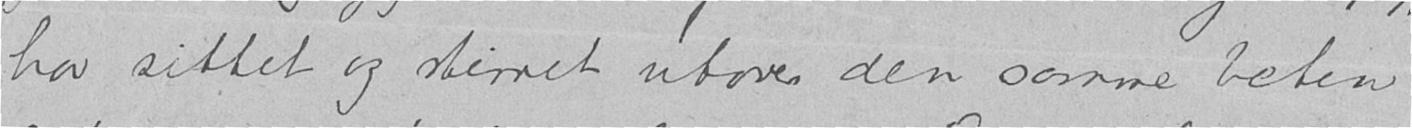har sittet og stirret utover den samme beten
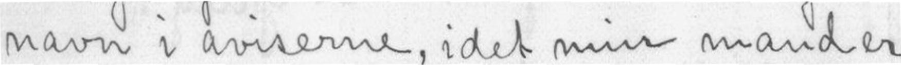navn i aviserne, idet min mand er
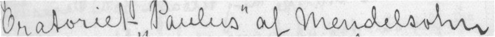Oratoriet Paulus af Mendelsohn
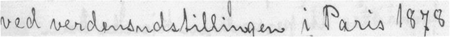ved verdensudstillingen i Paris 1878
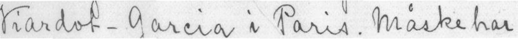Viardot-Garcia i Paris. Måske har
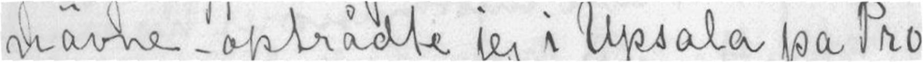nävne - optrådte jeg i Upsala på Pro-
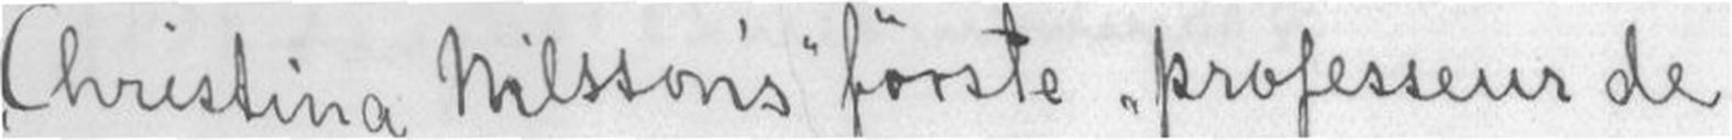Christina Nilsson's første professeur de
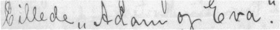billede Adam og Eva. -
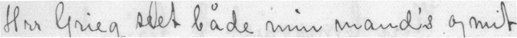Hrr Grieg seet både min Mand's og mit
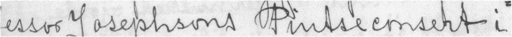fessor Josephsons Pintseconsert i
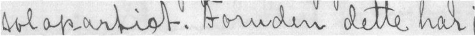solopartiet. Foruden dette har
